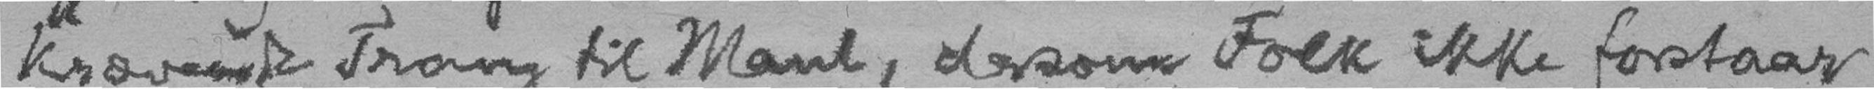krævende Trang til Maul, dersom Folk ikke forstaar
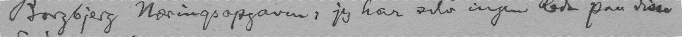Borgbjerg Næringsopgaven; jeg har selv ingen Rede paa disse
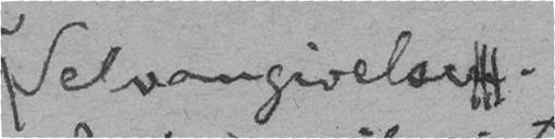Selvangivelsen.
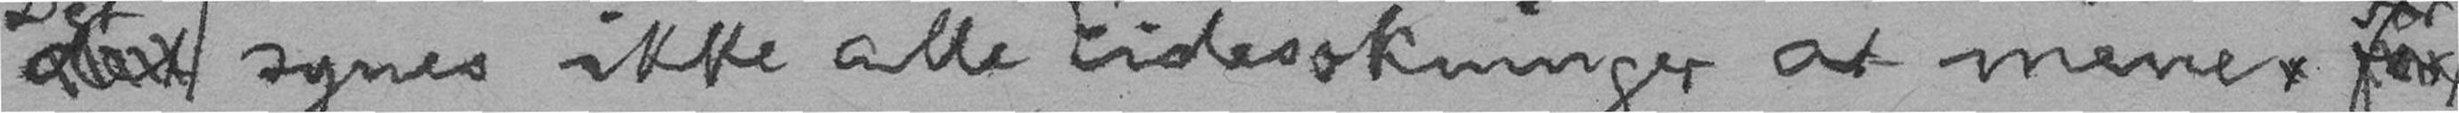det synes ikke alle Eidesokninger at mene, for
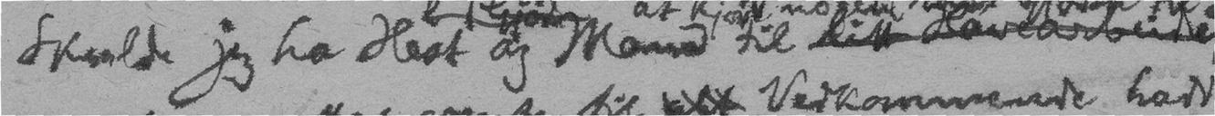Skulde jeg ha Hest og Mand til litt Havearbeide
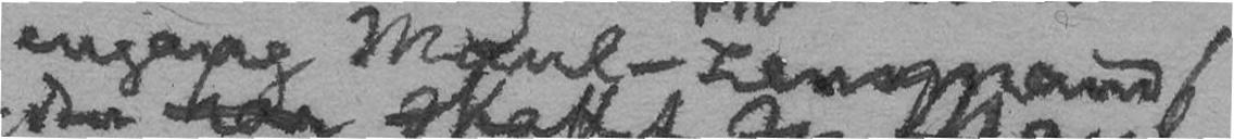engang Maul-Lensmand
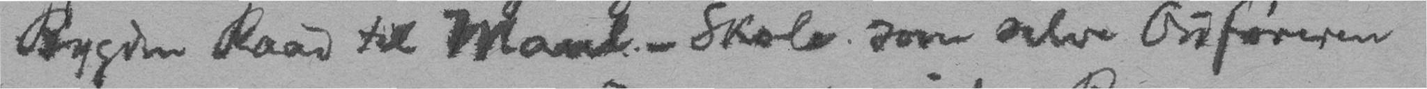Bygden Raad til Maul-Skole som selv Ordføreren
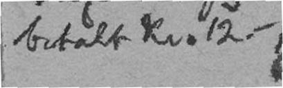betalt Kr. 12. -
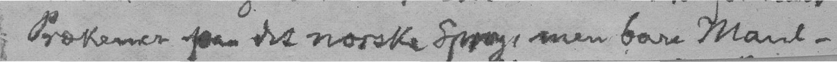Prækener paa det norske Sprog, men bare Maul-
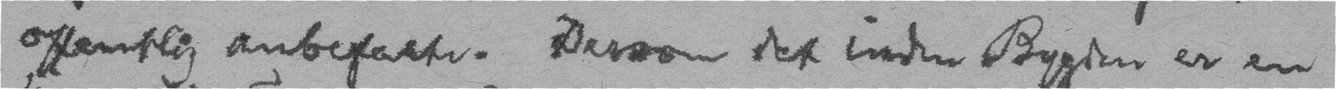offentlig anbefalte. Dersom det inden Bygden er en
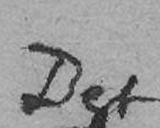Det
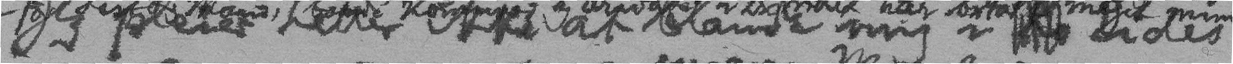Jeg pleier heller ikke at blande mig i der de des
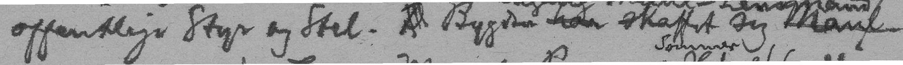offentlige Styr og Stel. D Bygden har skaffet sig Maul
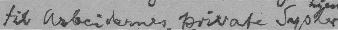til Arbeidernes private Sysler
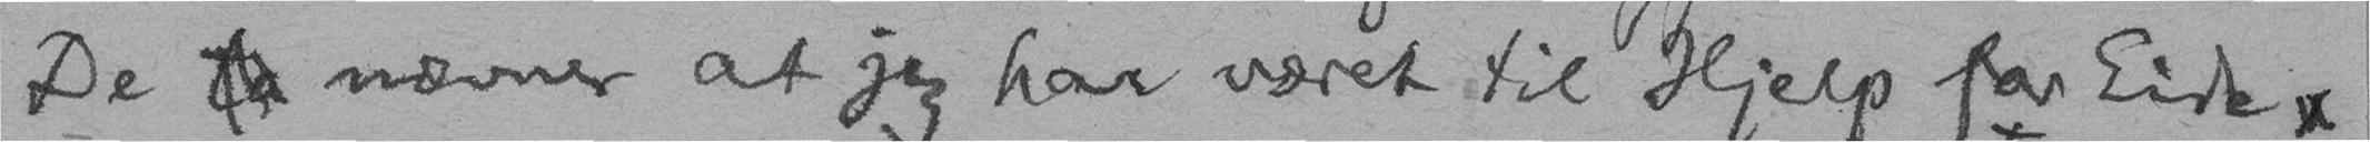De ta nævner at jeg har været til Hjelp for Eide,
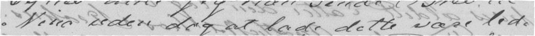Nina uden dog at lade dette være led-
